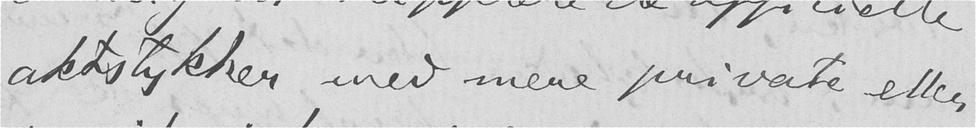aktstykker med mere private eller
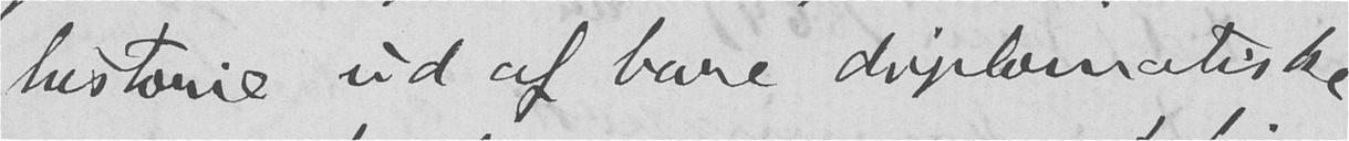historie ud af bare diplomatiske
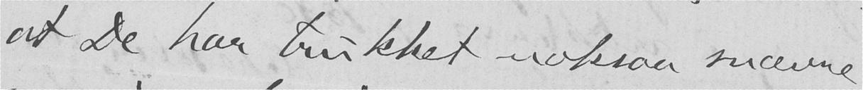at De har trukket noksaa snævre
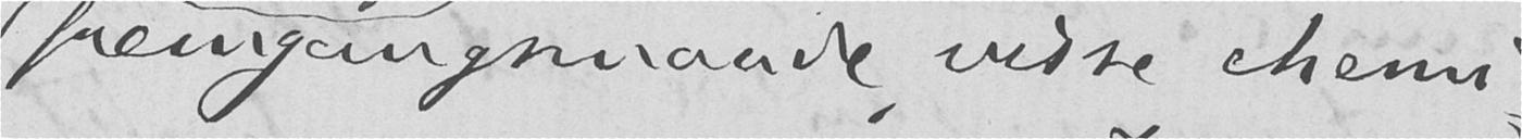fremgangsmaade, visse chemi-
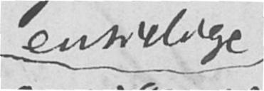ensidige
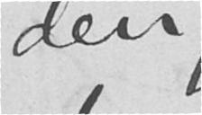den
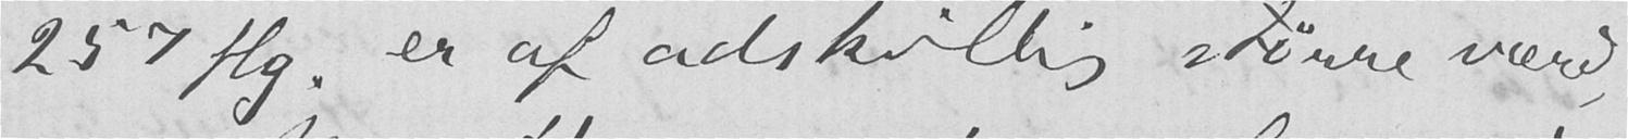257 flg. er af adskillig større værd,
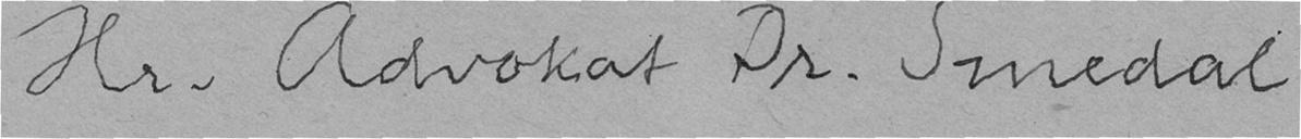Hr. Advokat Dr. Smedal
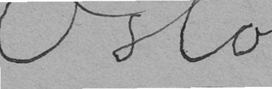Oslo
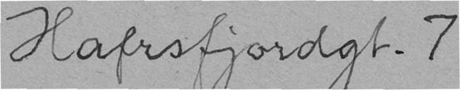Hafrsfjordgt. 7
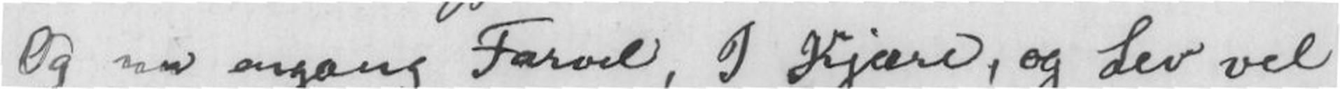Og nu engang Farvel, I Kjære, og Lev vel
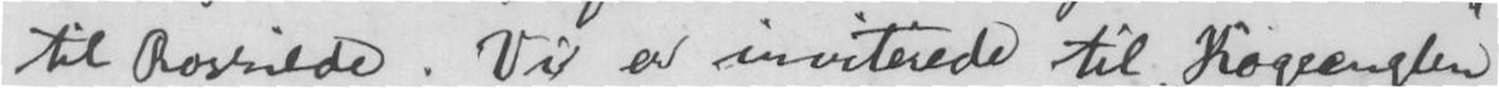til Roskilde. Vi er inviterede til Kogeenglen
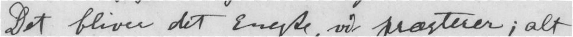Det bliver det Eneste, vi præsterer; alt
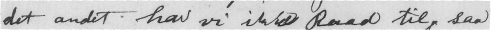det andet har vi ikke Raad til, saa
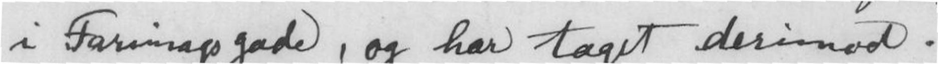i Farimagsgade, og har taget derimod.
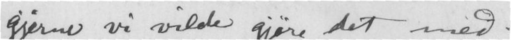gjerne vi vilde gjøre det med.
